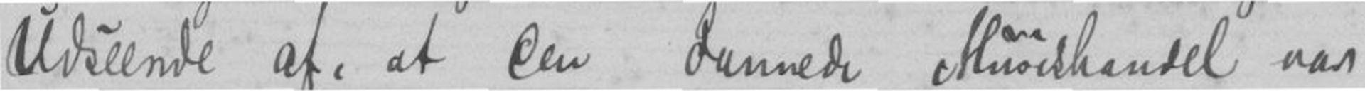Udseende af, at den dannede Musikhandel var
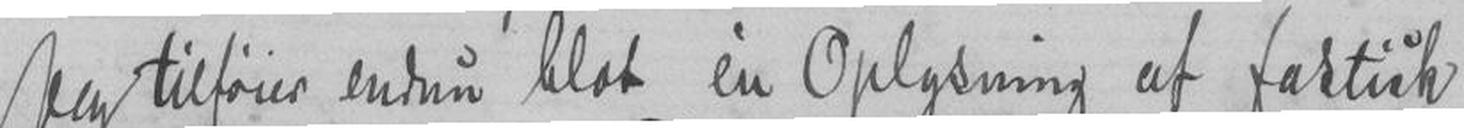Jeg tilføier endnu bløt en Oplysning af faktisk
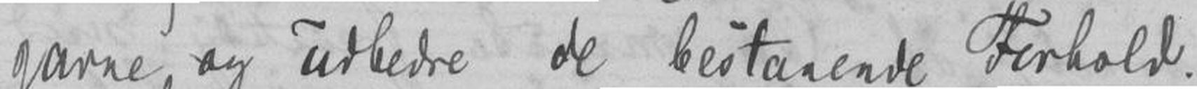garne og udbedre de bestaaende forhold.
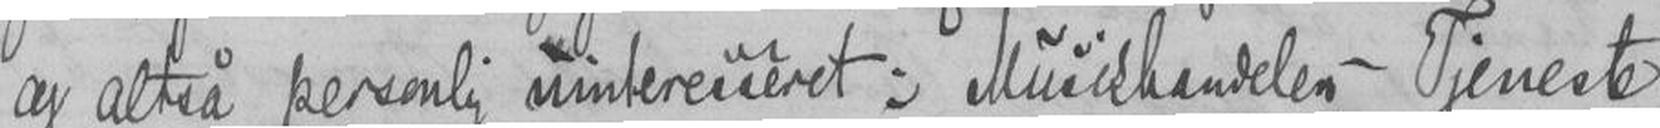og altså personlig uinteressert; musikhandelen - Tjeneste
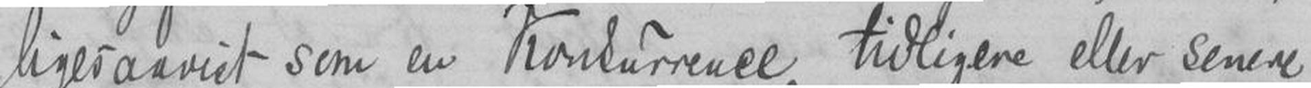ligesaavist som en Konkurrentt tidligere eller senere
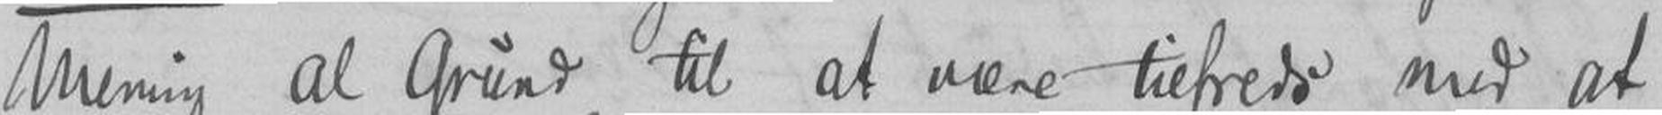Mening al grund til at være tilfreds med at
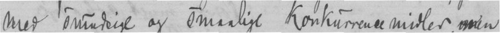med smudsige og smaalige Konkurrancemidler, men
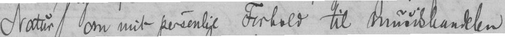Natur om mit personlige forhold til Musikhandelen,
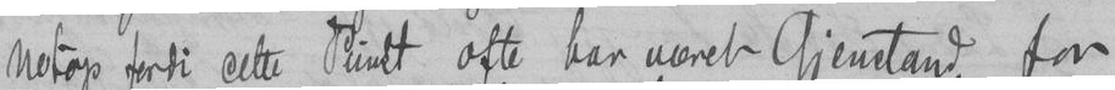netop fordi dette Punkt ofte har været Gjenstand for
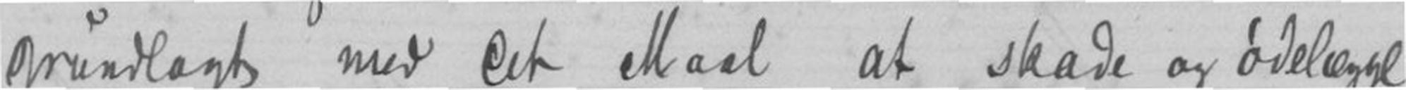grundlagt med det Maal at skade og ødelegge,
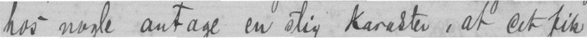hos nogle antage en stig Karakter, at fik
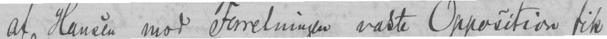af Hansen mod Forretninger vakte Opposition fik
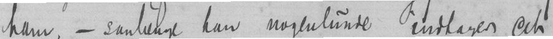ham, - saalaange han nogenlunde indtager det
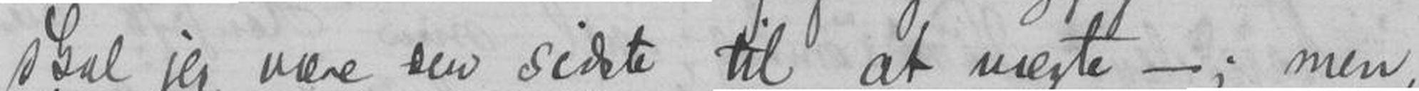skal jeg være den sidste til at negte-, men,
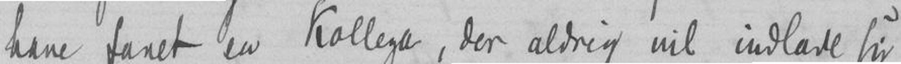have faaet en Kollega, der aldrig vil indlade sig
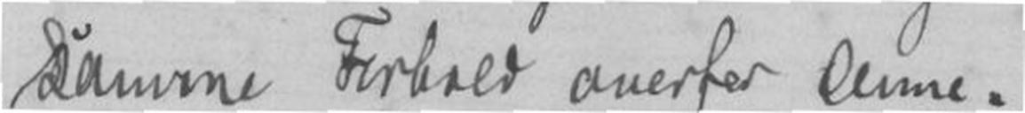samme Forhold overfor denne.
